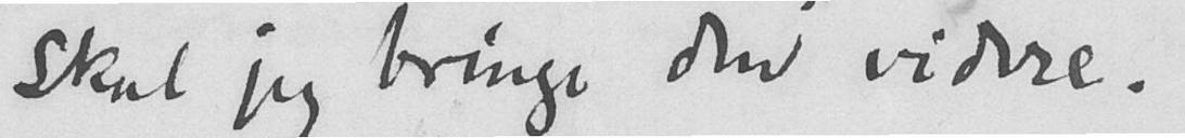skal jeg bringe den videre.
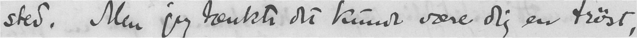sted. Men jeg tænkte det kunde være dig en trøst,
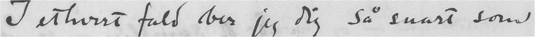I ethvert fald ber jeg dig så snart som
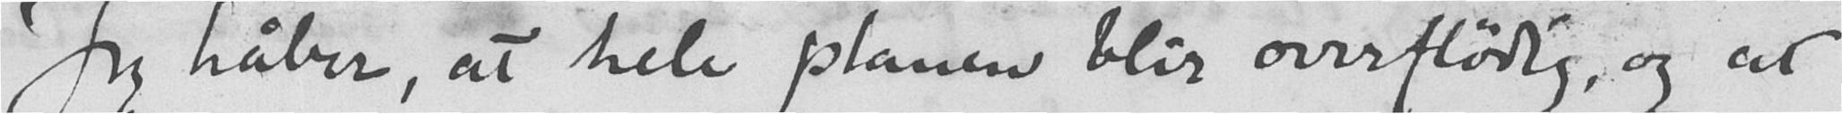Jeg håber, at hele planen blir overflødig, og at
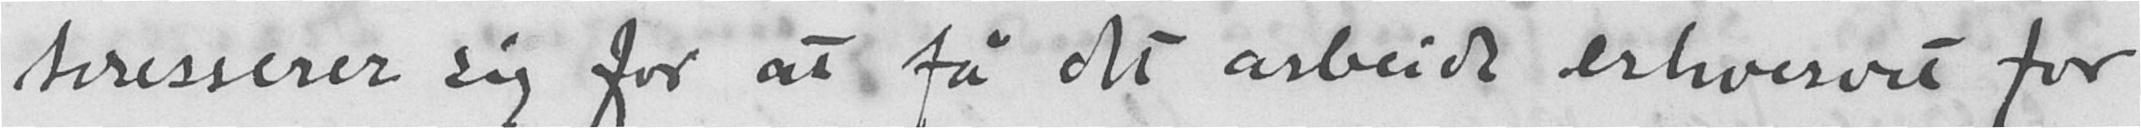teresserer sig for at få det arbeidet erhvervet for
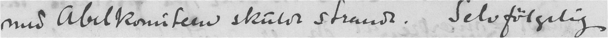med Abelkomiteen skulde strande. Selvfølgelig
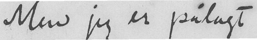Men jeg er pålagt
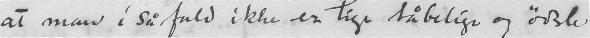at man i så fald ikke er lige tåbelige og ødsle
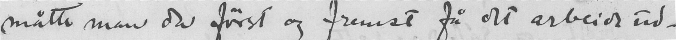måtte man da først og fremst få det arbeidet ud-
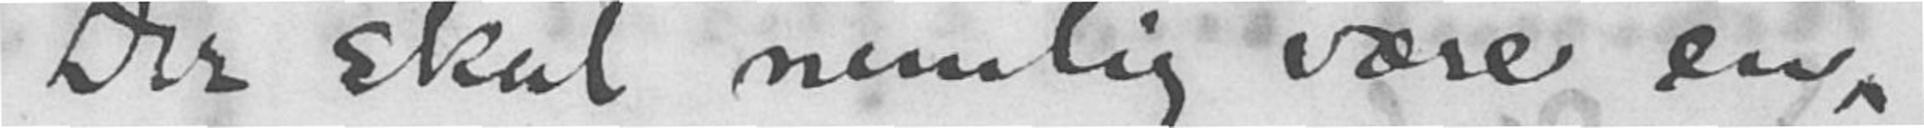Der skal nemlig være en
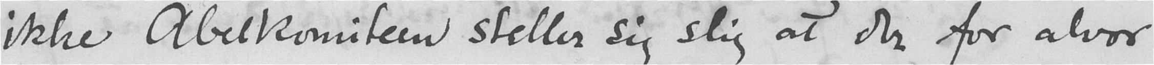ikke Abelkomiteen steller sig slig at der for alvor
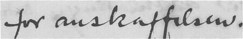for anskaffelsen.
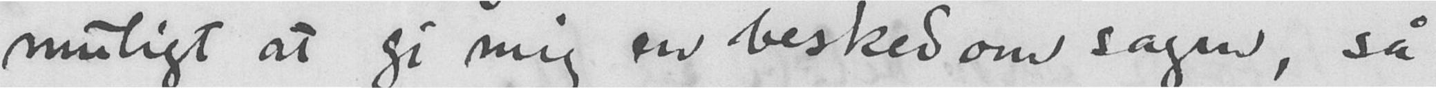muligt at gi mig en besked om sagen, så
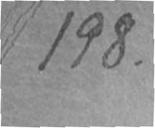198.
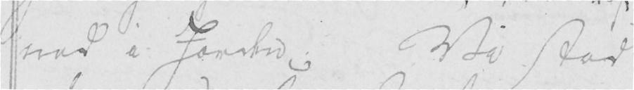ned i Jorden. Vi stod
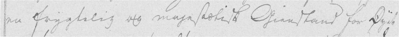en frygtelig og majestætisk Gienstand for Øyet
